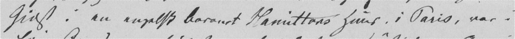Gjæst i en engelsk Baronet Hamittons Huus, i Paris, var i
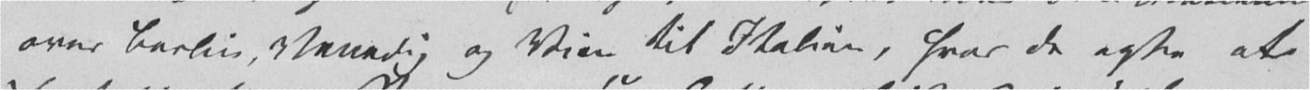over Berlin, Venedig og Wien til Italien, hvor de agte at
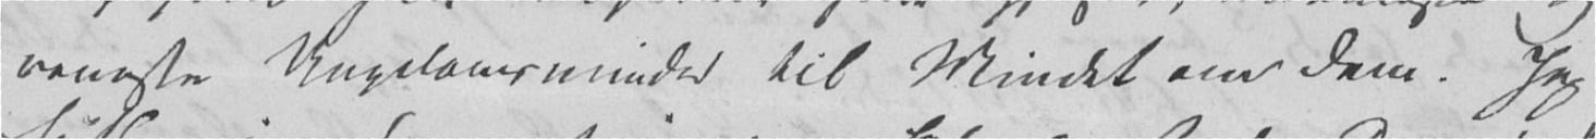reneste Ungdomsminder til Mindet om Dem. Jeg
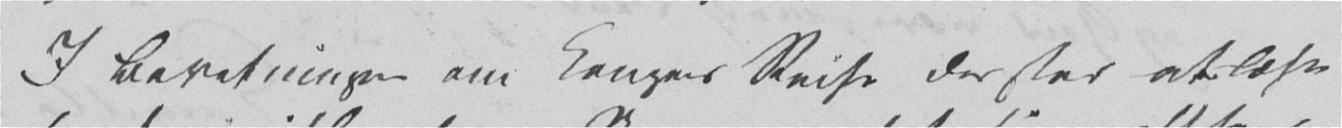I Beretningen om Kongens Reise der stod at læse
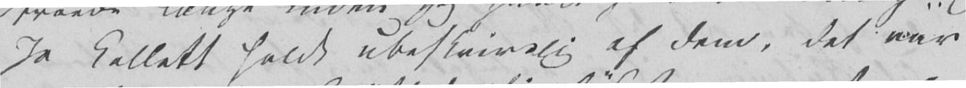Ja Collett holdt ubeskrivelig af Dem, det var
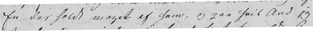En der holdt meget af ham, og paa hvis Ord jeg
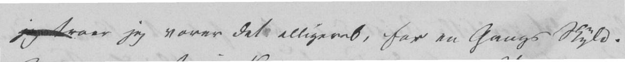jeg troer jeg vover det alligevel, for en Gangs Skyld.
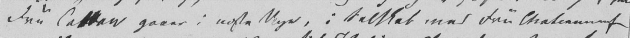Fru Colban gaaer i næste Uge, i Selskab med Fru Chateauneuf
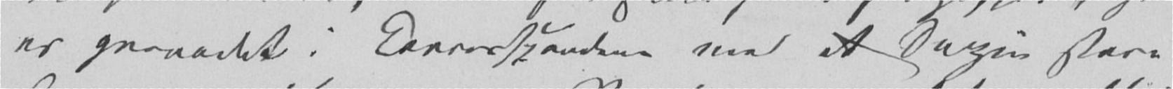er geraadet i Korresspondence med et Duzin store
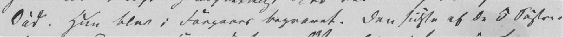Død. Hun blev i Forgaars begravet. Den sidste af de 5 Søstre.
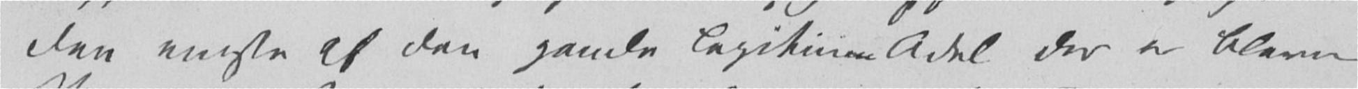den eneste af den gamle legitime Adel der er bleven
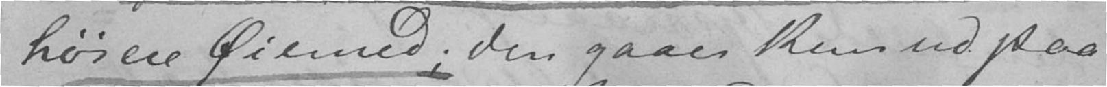høiere Øiemed; den gaaer kun ud paa
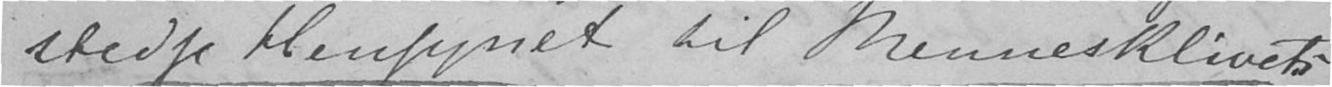stedse Hensynet til Menneskelivets
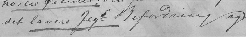det lavere Jegs Befordring og
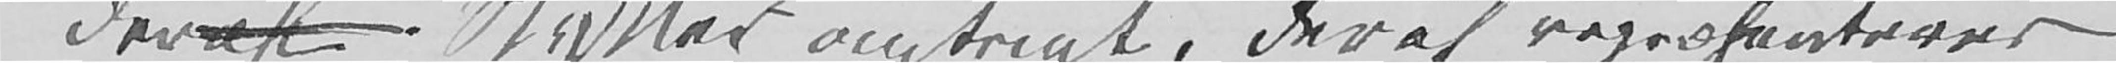deraf Stykker omtrent, deraf repræsenterer
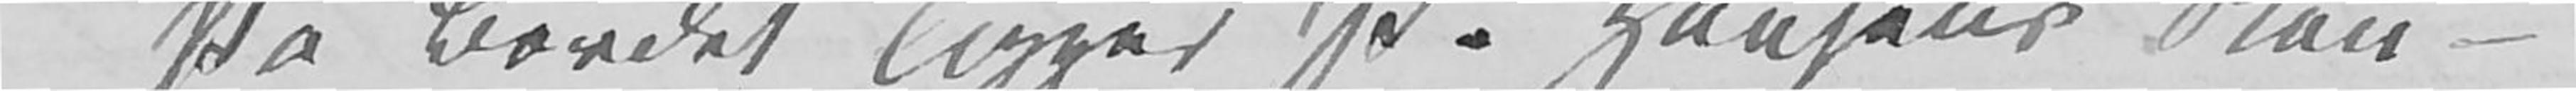På Bordet ligger P. Hansens «Skan¬
